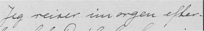Jeg reiser imorgen efter-
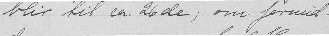blir til ca. 26de; om formid-
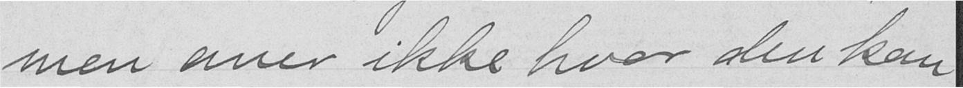men aner ikke hvor den kan
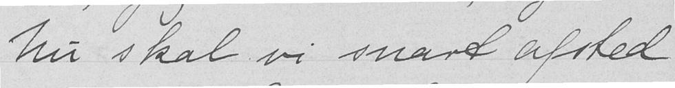Nu skal vi snart afsted
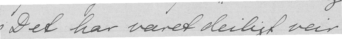Det har været deiligt veir
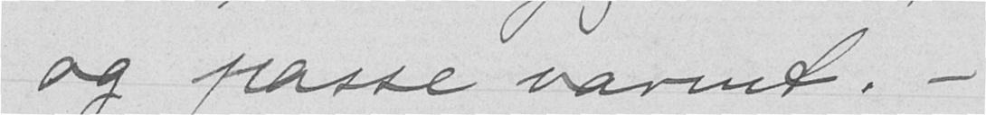og passe varmt. -
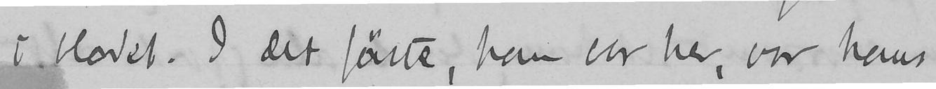i blodet. I det første, han var her, var hans
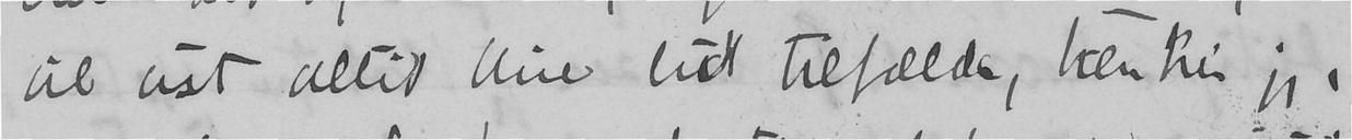vil vist altid blive lidt tilfælde, tænker jeg ,
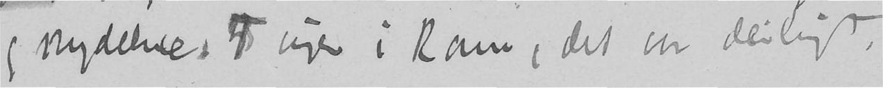og nydelsees, 4 uger i Rom, det er deiligt.
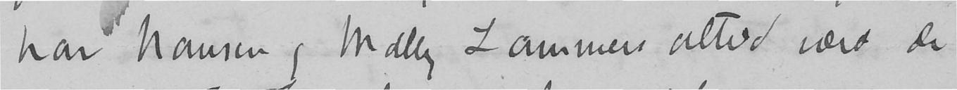har Nansen og Mally Lammers altid vært de
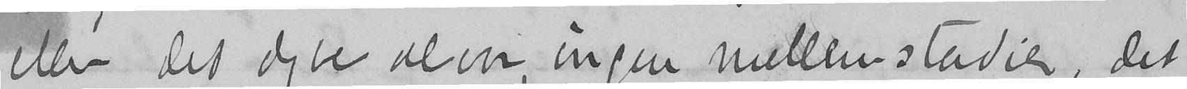eller det dybe alvor, ingen mellemstadier, det
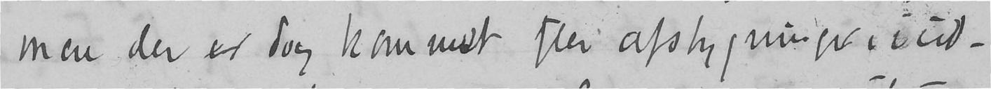men der er dog kommet flere afskygninger i ind-
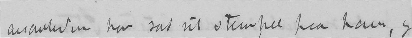arsomheden har sat sit stempel paa ham, og
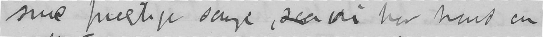sine pregtige sange, saa vi har havt en
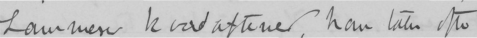Lammers kvadaftener, han taler ofte
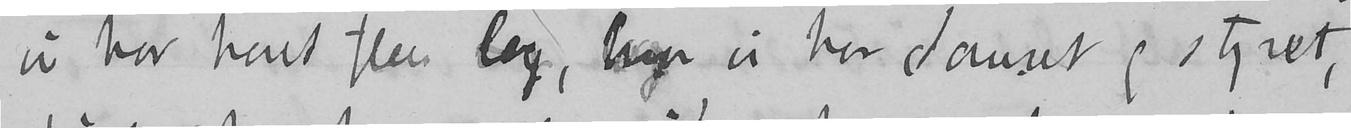vi har havt flere lag, hvor vi har danset og styret,
